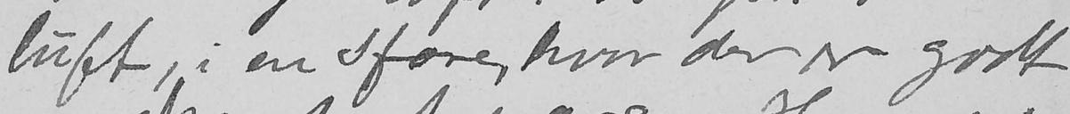luft, i en sfære, hvor der er godt
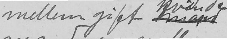mellem gift mand
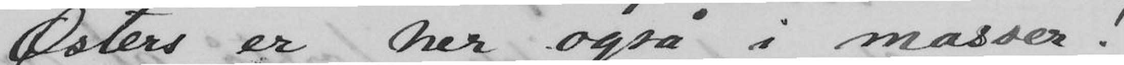Østers er her også i masser!
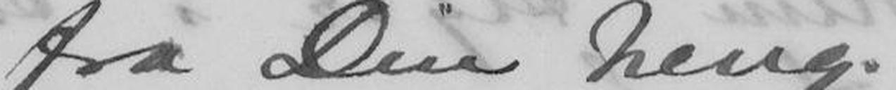fra Din heng.
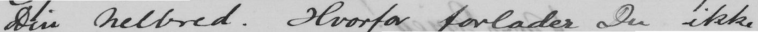Din helbred. Hvorfor forlader Du ikke
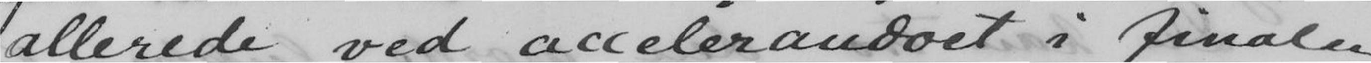allerede ved accelerandoet i finalen.
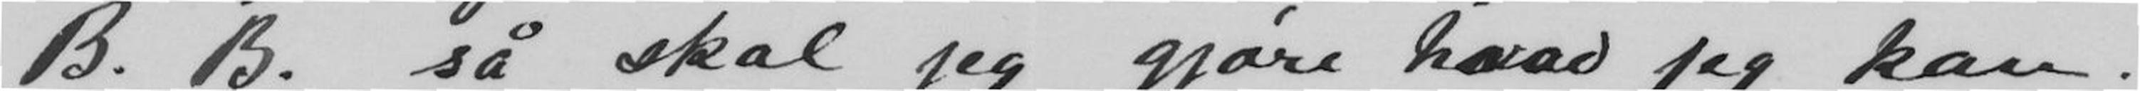B.B. så skal jeg gjøre hvad jeg kan.
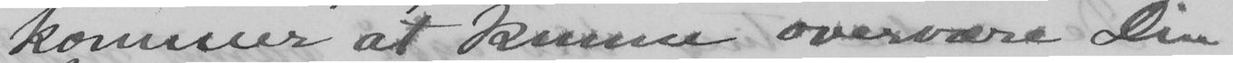kommer at kunne overvære Din
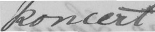koncert.
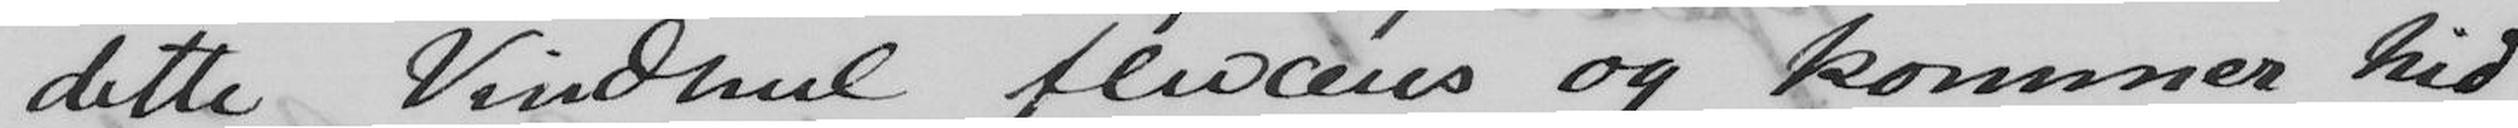dette Vindhul fluxens og kommer hid
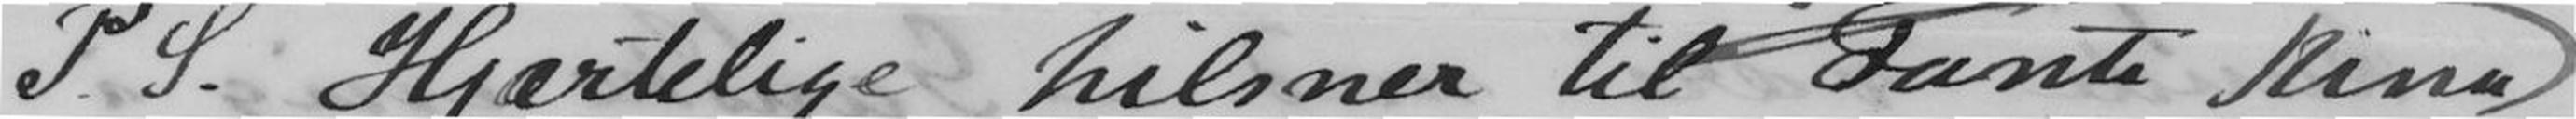P.S. Hjærtelige hilsner til Tante Nina
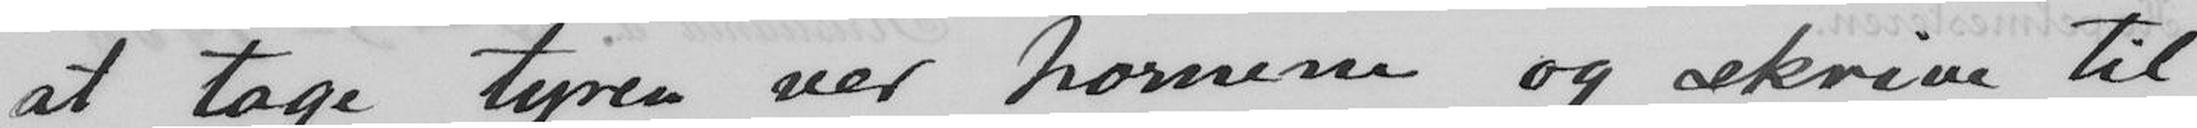at tage tyren ved hornene og skrive til
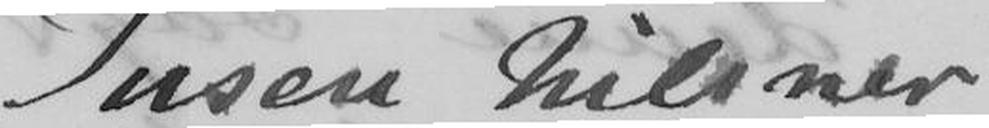Tusen hilsner
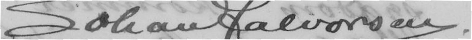Johan Halvorsen.
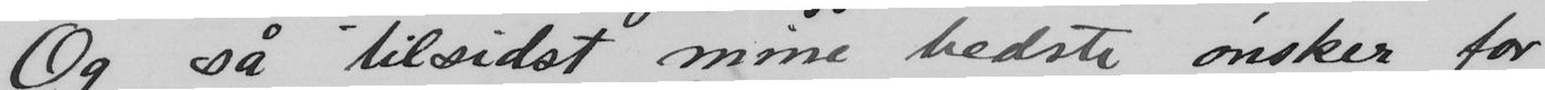Og så tilsidst mine bedste ønsker for
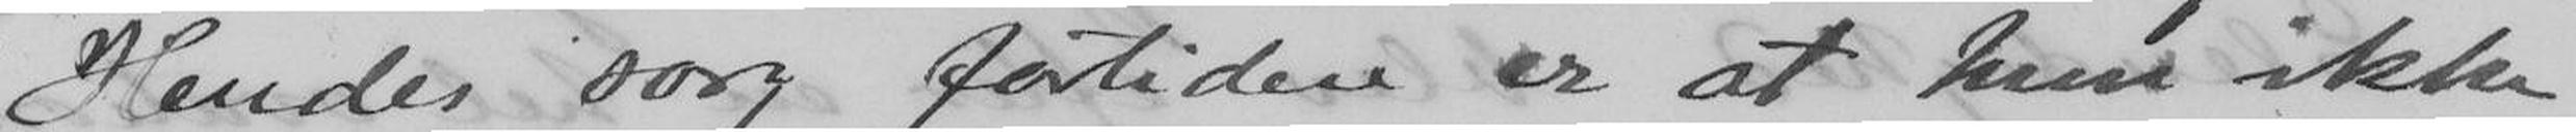Hendes sorg fortiden er at hun ikke
